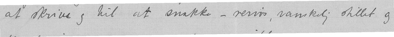at skrive og til at snakke – nervøs, vanskelig stillet og
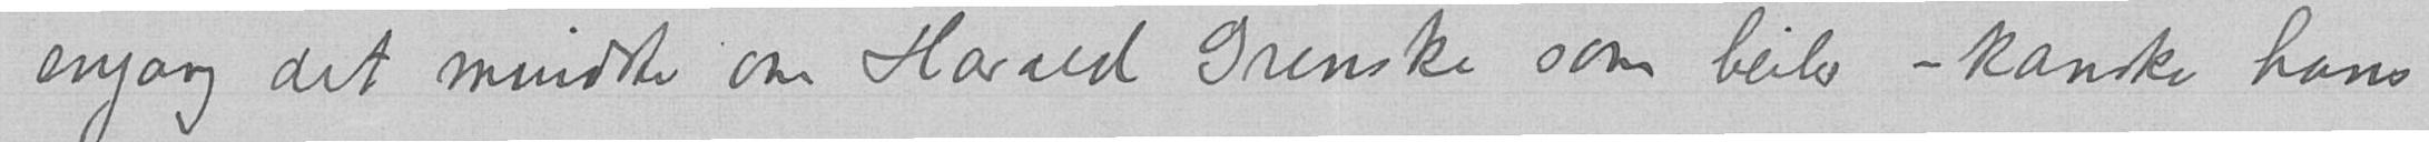engang det mindste om Harald Grenske som beiler – kanske hans
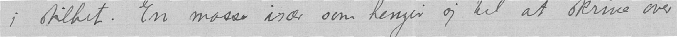i stilhet. En masse især som henger sig til at skrive over
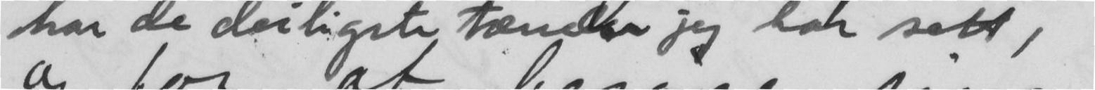har de deiligste tænder jeg har sett,
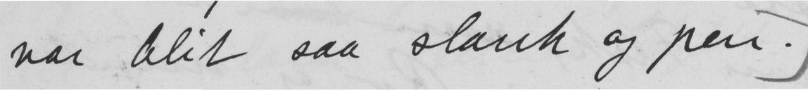var blit saa slank og pen.
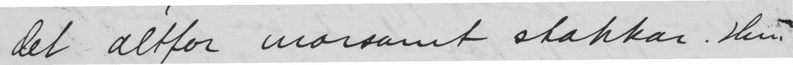det altfor morsomt stakkar. Hun
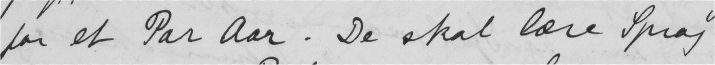for et Par Aar. De skal lære Sprog
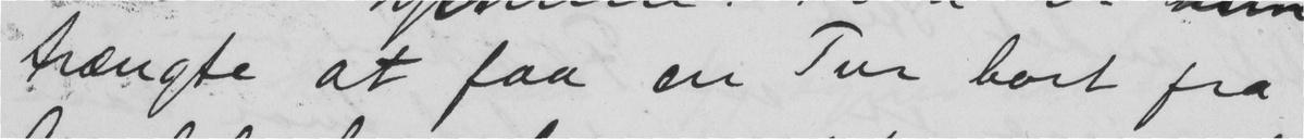trængte at faa en Tur bort fra
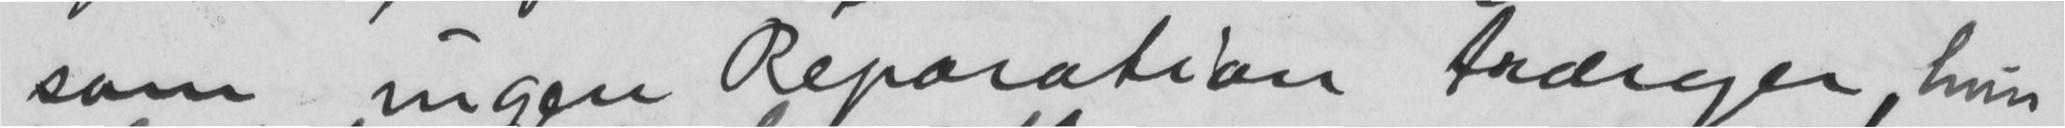som ingen Reparation trærger, hun
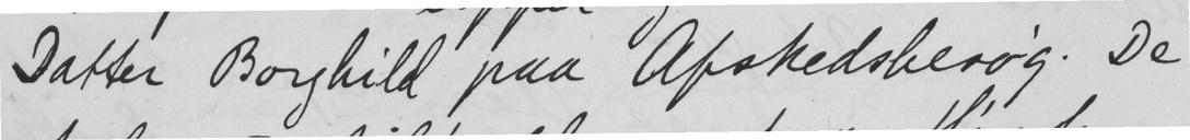Datter Borghild paa Afskedsbesøg. De
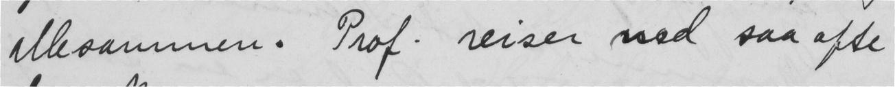allesammen. Prof. reiser ned saa ofte
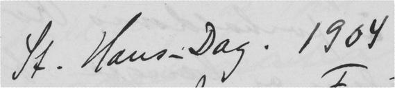St. Hans-Dag. 1904
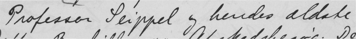Professor Seippel og hendes ældste
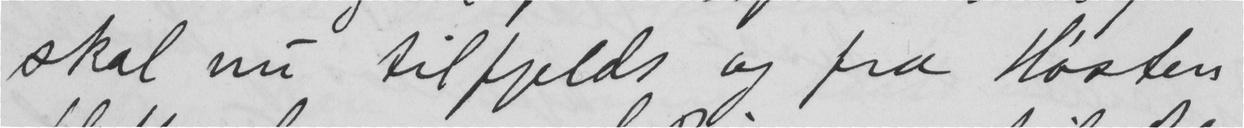skal nu tilfjelds og fra Høsten,
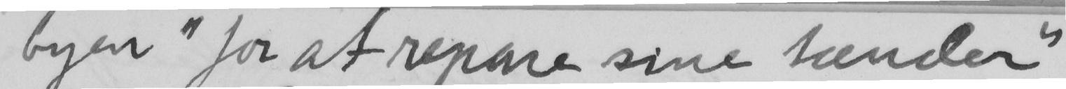byen "for at repare sine tænder"
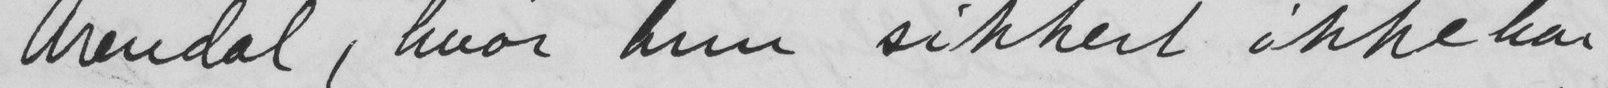Arendal, hvor hun sikkert ikke har
